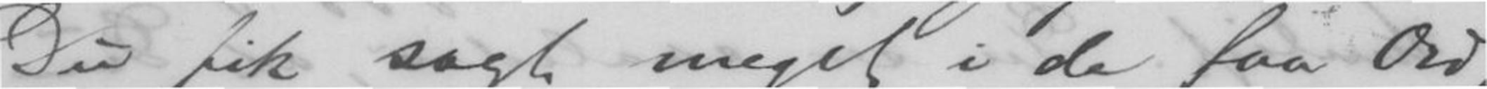Du fik sagt meget i de faa Ord,
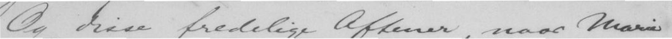Og disse fredelige Aftener, naar Marie
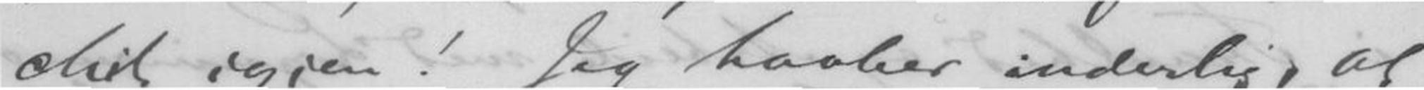chit igjen! Jeg haaber inderlig, at
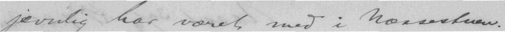jevnlig har været med i Næssestuen.
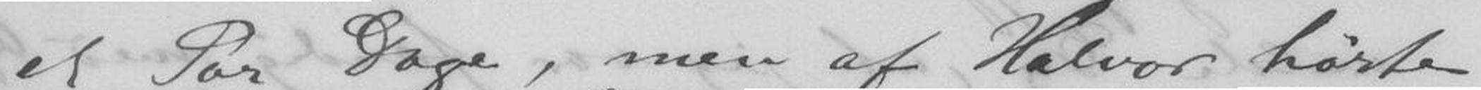et Par Dage, men af Halvor hørte
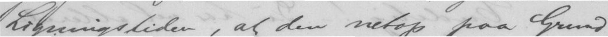Ligningstiden, at den netop paa Grund
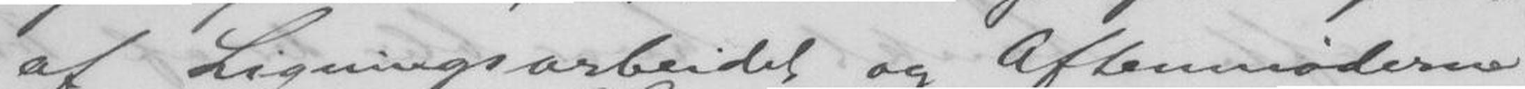af Ligningsarbeidet og Aftenmøderne
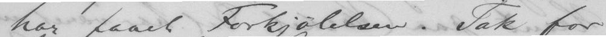har faaet Forkjølelsen. Tak for
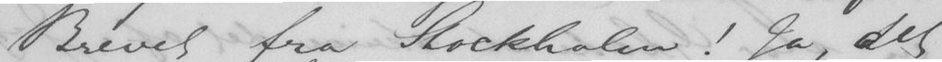Brevet fra Stockholm! Ja, det
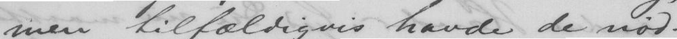men tilfældigvis havde de nød-
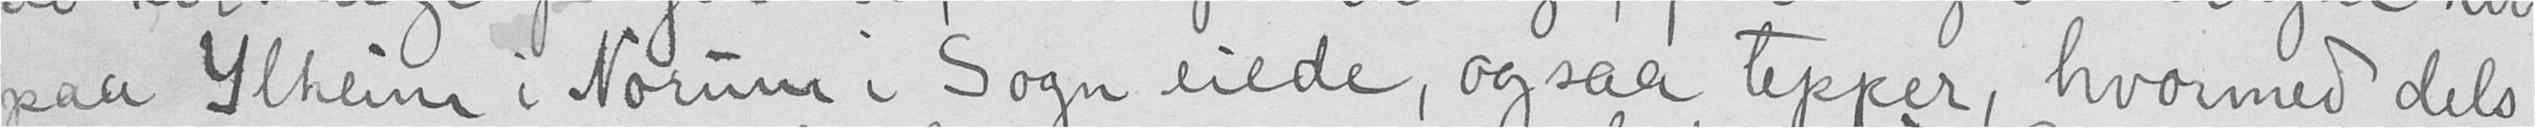paa Ylheim i Norum i Sogn eiede, ogsaa tepper, hvormed dels
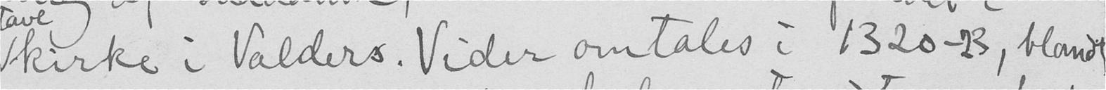kirke i Valders. Vider omtales i 1320-23, blandt
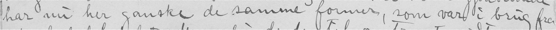har nu her ganske de samme former, som var i brug fra
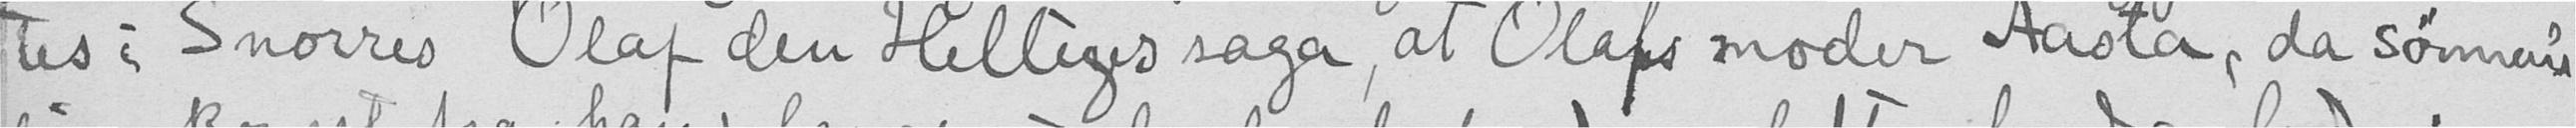tes i Snorres Olaf den Helliges saga, at Olafs moder Aasta, da sønnens
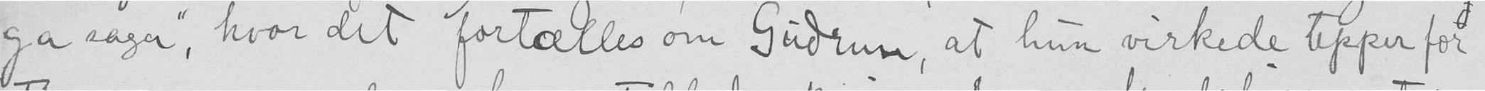ga saga", hvor det fortælles om Gudrun, at hun virkede tepper for
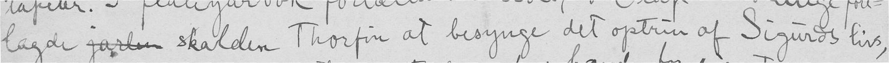lagde  skalden Thorfin at besynge det optrin af Sigurds livs,
